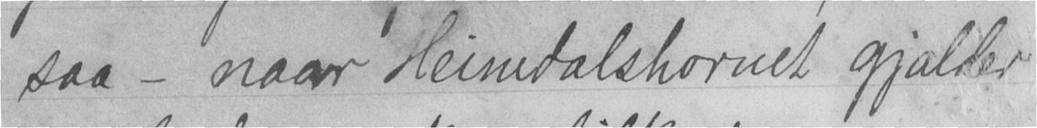saa - naar Heindalshornet gjalder
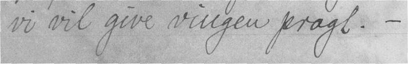vi vil give vingen pragt. -
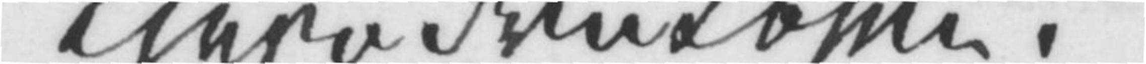Kjere Fru Ibsen!
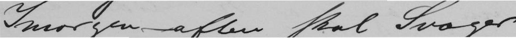Imorgenaften skal Svoger
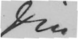Din
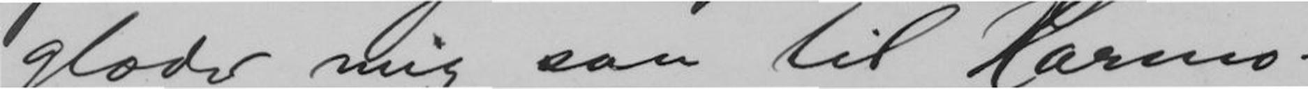glæder mig saa til Harmo-
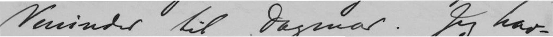Veninder til Dagmar. Jeg hav-
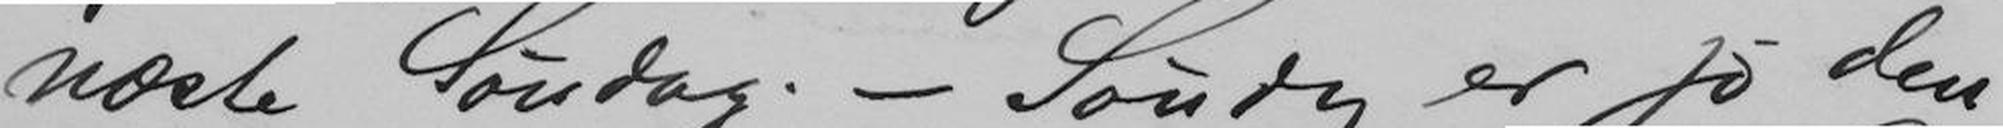næste Søndag. - Søndag er jo den
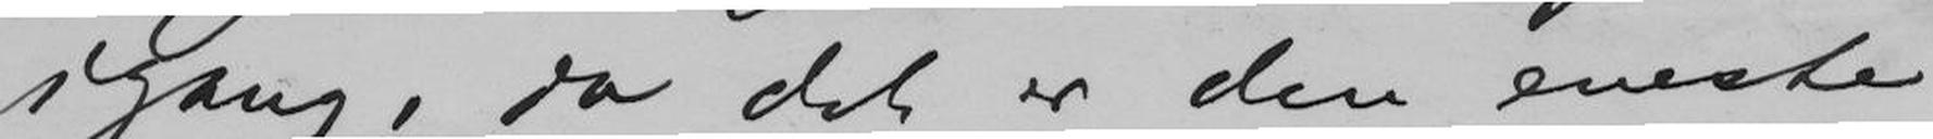igang, da det er den eneste
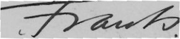Frants.
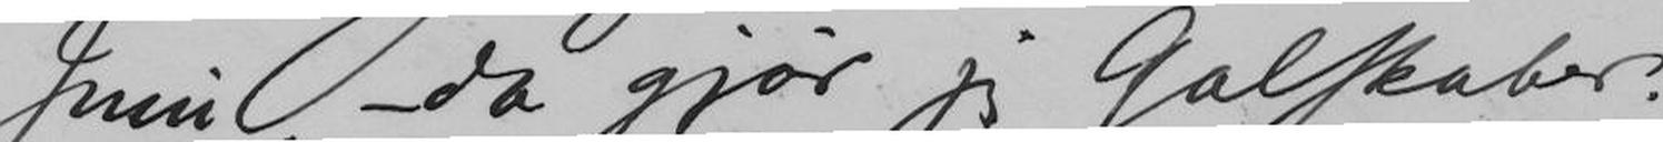Juni, da gjør jeg Galskaber.
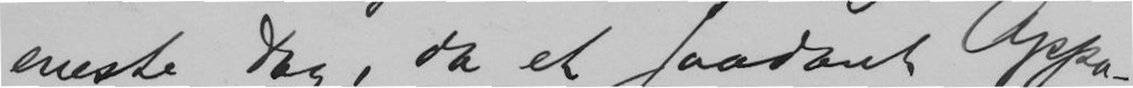eneste Dag, da et saadant Appa-
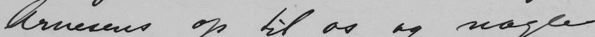Arnesens op til os og nogle
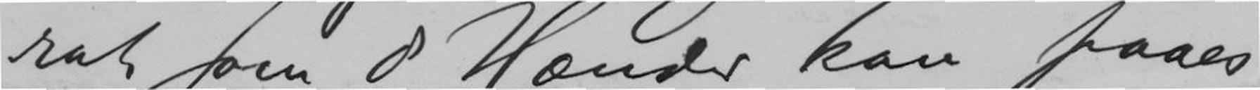rat som 8 Hænder kan faaes
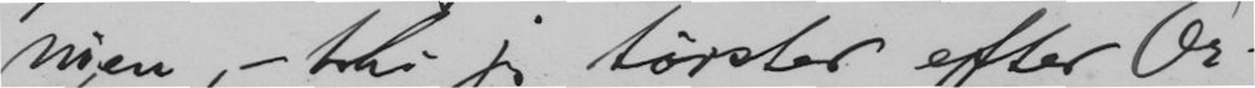nien, - thi jeg tørster efter Or-
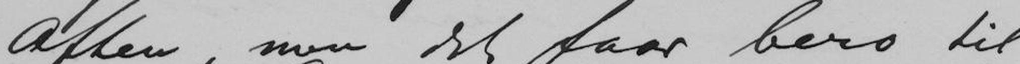Aften, men det faar bero til
